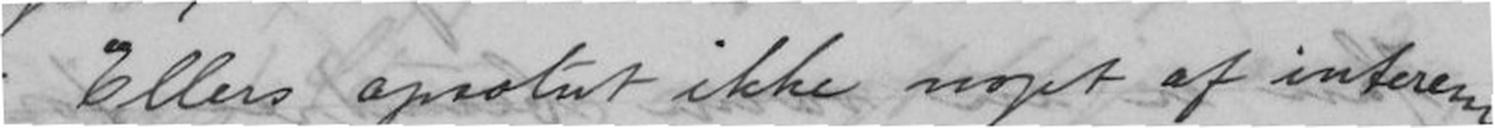Ellers apsolut ikke noget af interesse
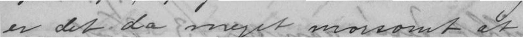er det da meget morsomt at
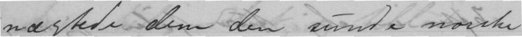nægtede dem den sunde norske
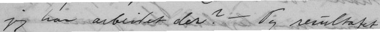jeg har arbeidet der? - Og resultatet
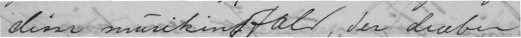disse musikindfald, der dræber
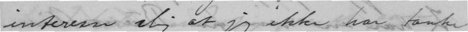interesse slig at jeg ikke har tanke
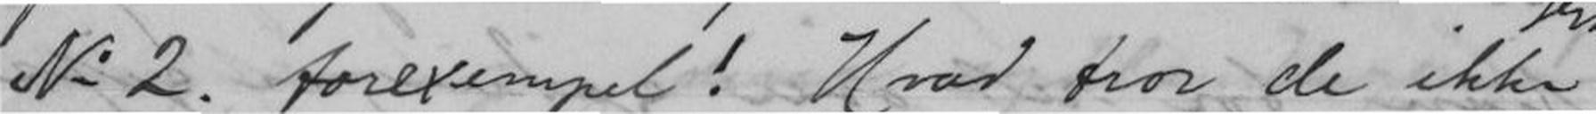N. 2. forexempel! Hvad tror de ikke
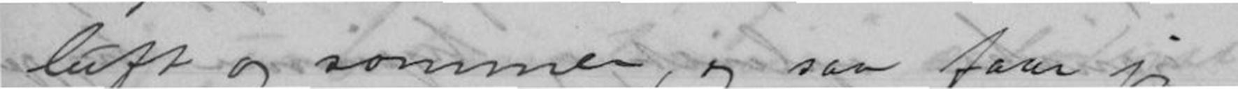luft og sommer, og saa faar jeg
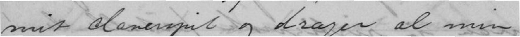mit claverspil og drager al min
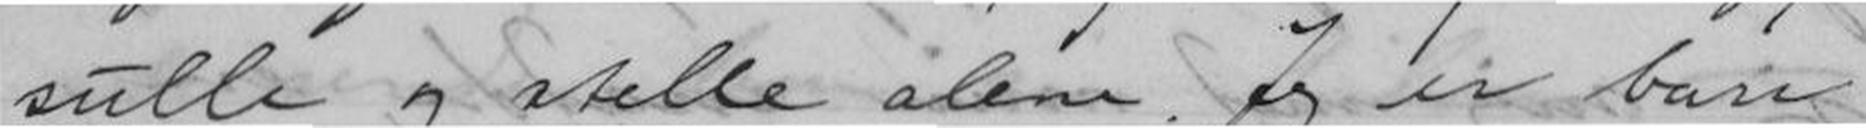sulle og stelle alene. Jeg er bare
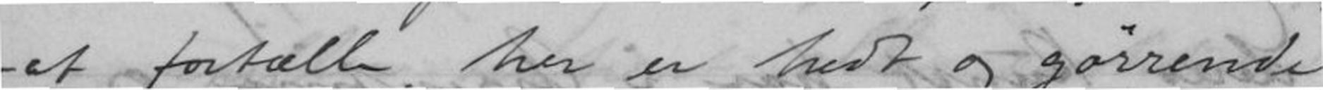at fortælle, her er hedt og görrende
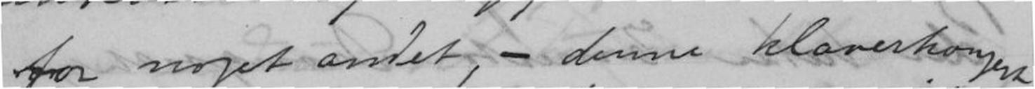for noget andet, - denne klaverkonzert
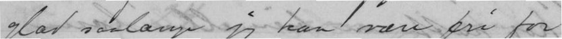glad saalænge jeg kan være fri for
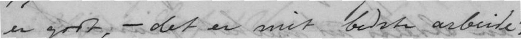er godt, - det er mit bedste arbeidet! -
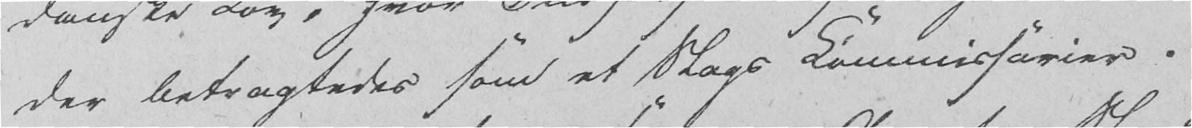der betragtedes som et Slags Kommissarier.
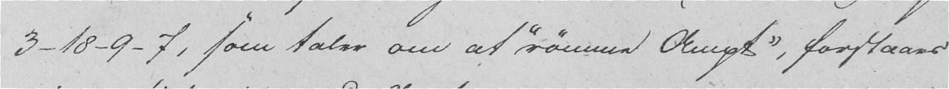3-18-9-7, som taler om at "rømme Ampt", forstaaes
